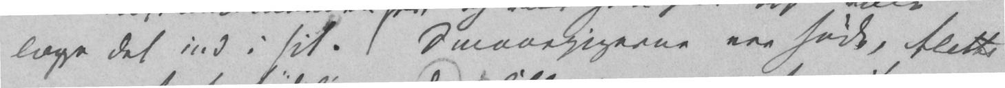lægge det ind i sit. Smaaepigerne ere søde, Alette
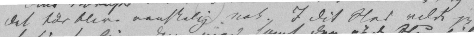det tør blive vanskelig nok. I Dit Sted vilde jeg
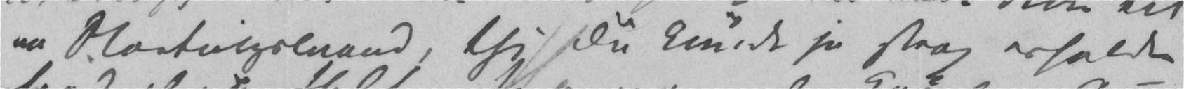en Stortingsmand, thi Du kunde jo strax erholde
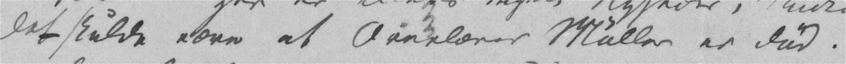det skulde være at Overlærer Müller er død.
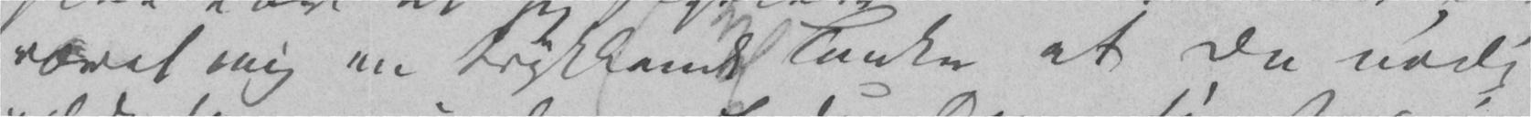været mig en trykkende Tanke at Du nødig
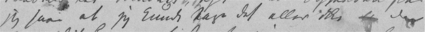jeg saa at jeg kunde tage det eller ikke da
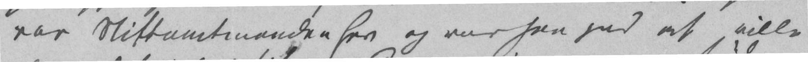var Stiftamtmanden her og var saa god at ville
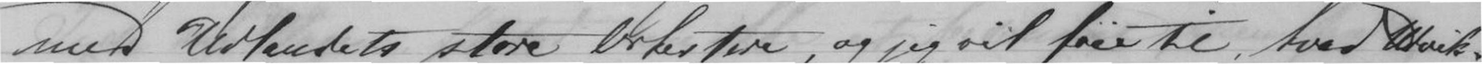med Udlandets store Orkestere, og jeg vil føie til, hvad Udvik-
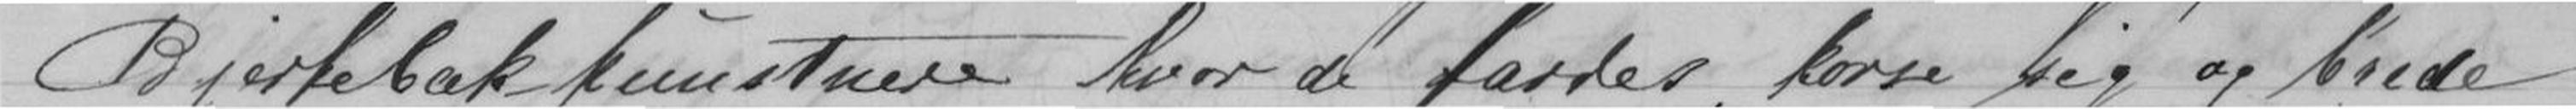Bjerkebækkunstnere hvor de færdes, korse sig og brede
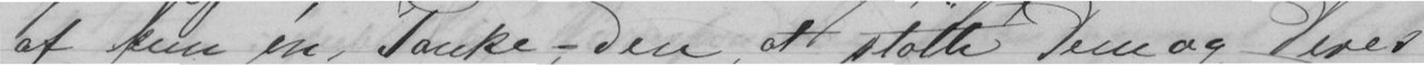af kun én Tanke - den, at støtte Dem og Deres
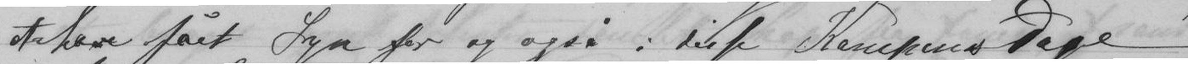at have fået Syn for og også i disse Kampens Dage
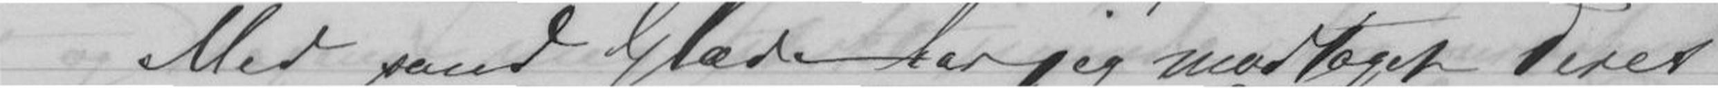Med sand Glæde har jeg modtaget Deres
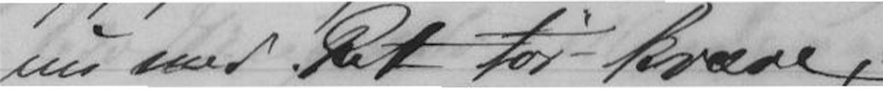nu med Ret tør kræve,
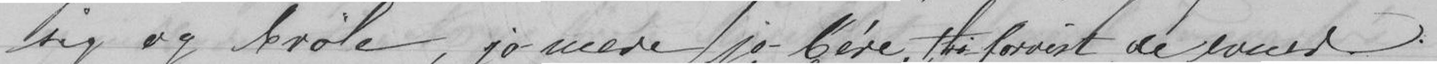sig og brøle, jo mere jo bére, thi forvist de evned
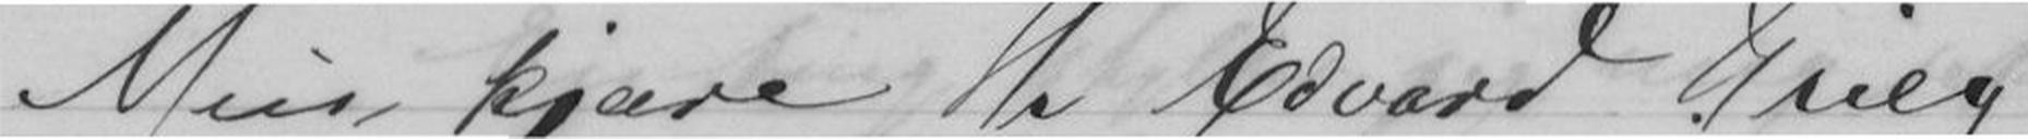Min kjære Hr Edvard Grieg
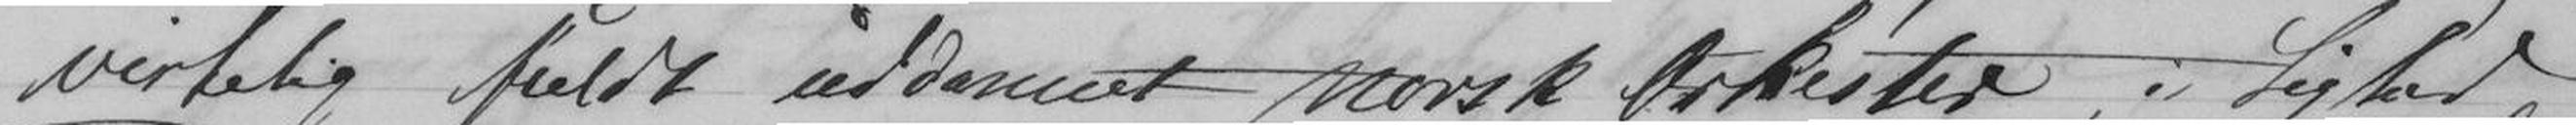virkelig fuldt uddannet norsk Orkester i Lighed
